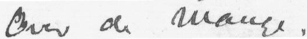Over de Mange.
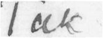Tak.
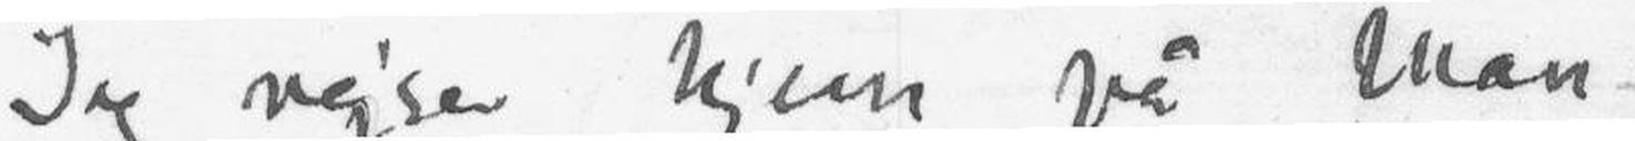Jeg rejser hjem på Man-
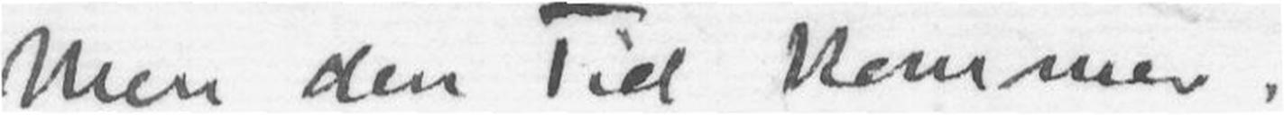Men den Tid kommer.
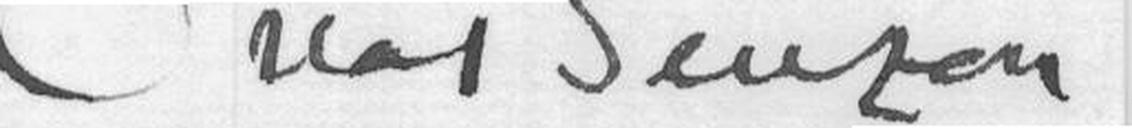Otto Benzon
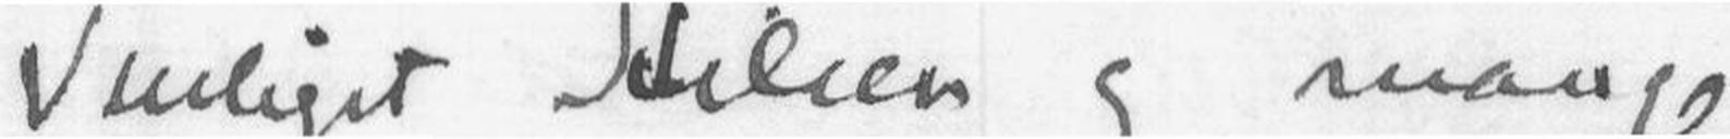Venligst Hilsen og mange
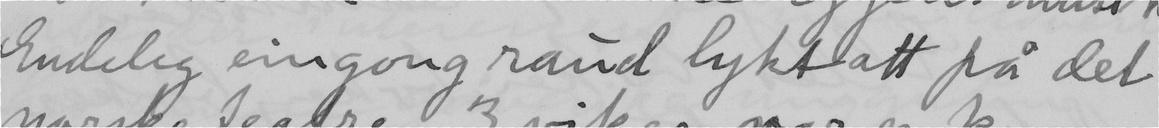Endeleg eingong raud lykt att på det
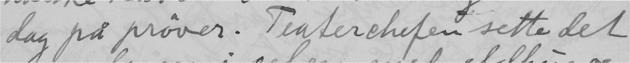dag på pröver. Teaterchefen sette det
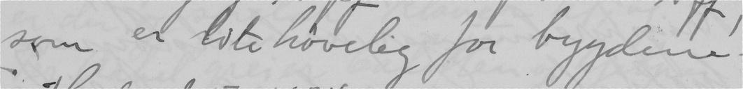som er lite hövelig for bygdene.
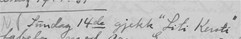Sundag 14de gjekk "Liti Kersti" i
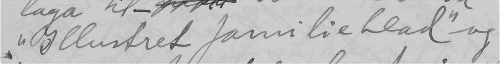"Illustrert familieblad" og
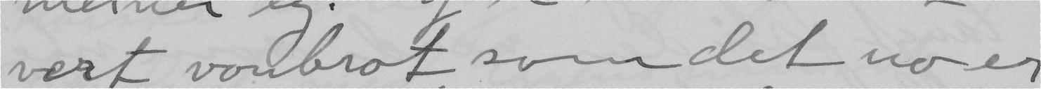vert vonbrot som det no er
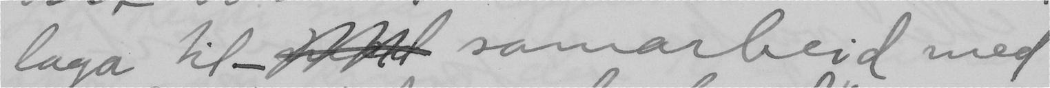laga til – samarbeid med
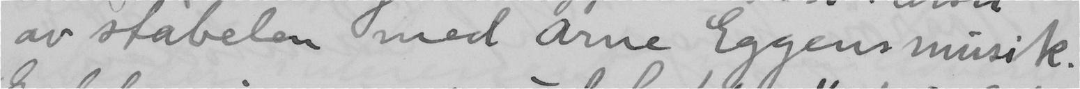av stabelen med Arne Eggens musik.
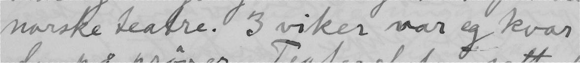norske teatre. 3 viker var eg kvar
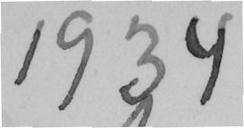1934
